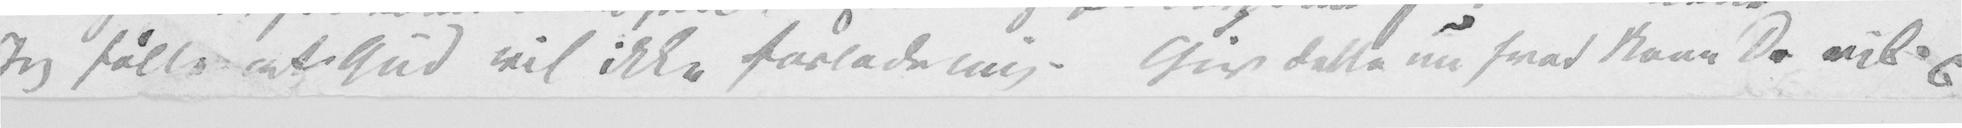Jeg følte at Gud vil ikke forlade mig. Giv dette nu hvad Navn De vil. C.
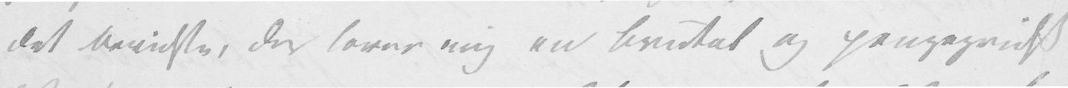det Bevidste, der lover mig en brutal og pengegridsk
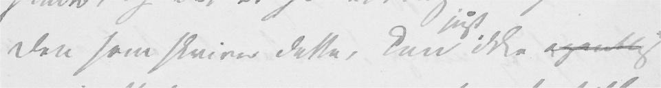Den som skriver dette, kan just ikke egentlig
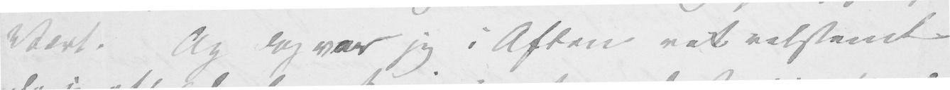Vært. Og dog var jeg i Aften ret velstemt
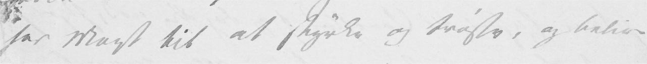har Magt til at styrke og trøste, og belive
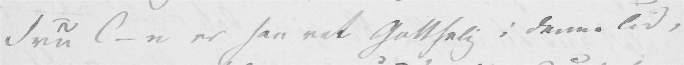Fru C-n er saa ret Gottselig i denne Tid,
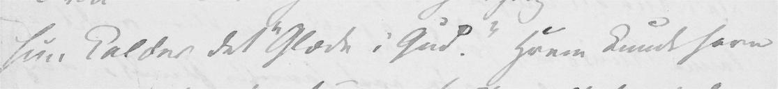hun kalder det «Glæde i Gud.» Hvem kunde have
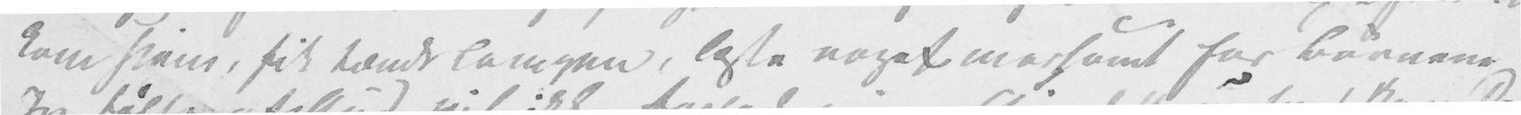kom hjem, fik tændt Lampen, læste noget morsomt for Børnene.
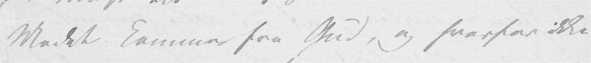Modet kommer fra Gud, og hvorfor ikke
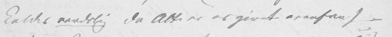kaldes verdslig da Alt er os givet ovenfra) –
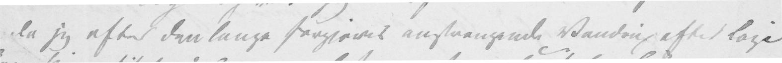da jeg efter den lange forgjæves anstrengende Vandring efter Logi
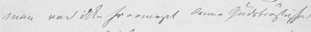Man ved ikke hvormeget denne Gudstrøstighed
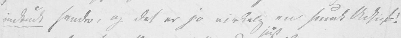indbudt hende, og det er jo virkelig en smuk Udsigt!
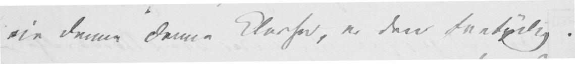eie denne denne Klarhed, er den tvetydig.
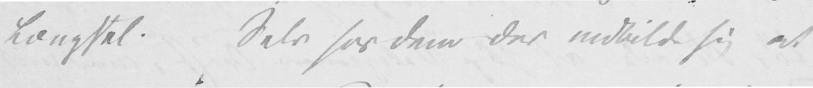Længsel. Selv for dem der indbilde sig at
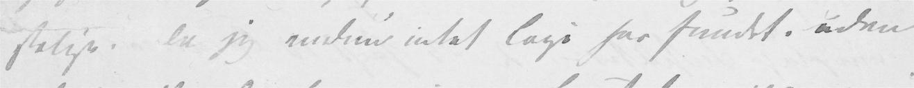stelige, da jeg endnu intet Logi har fundet. uden
